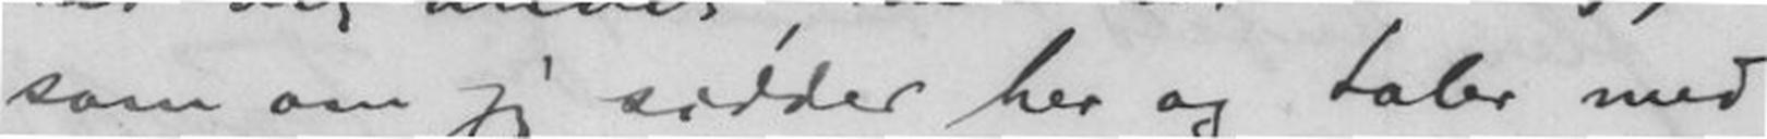som om jeg sidder her og taler med
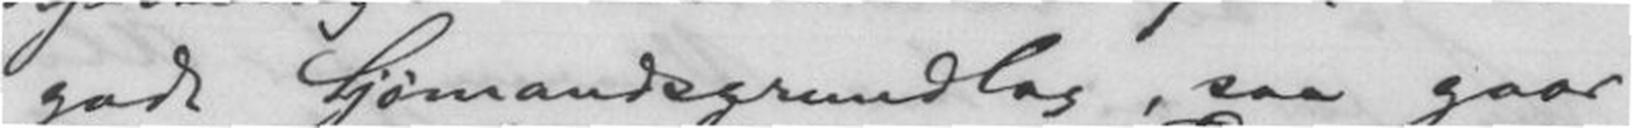godt Sjømandsgrundlag, saa gaar
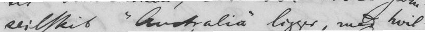seilskib "Australia" ligger, med hvil-
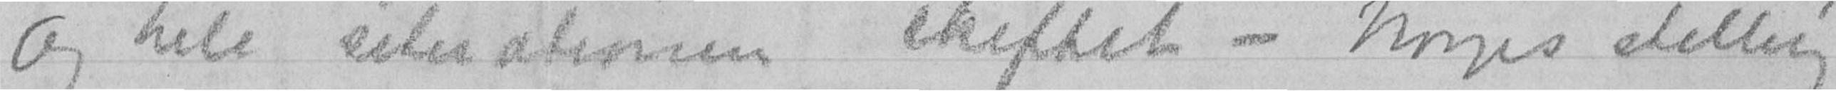Og hele situationen skiftet - Norges stilling
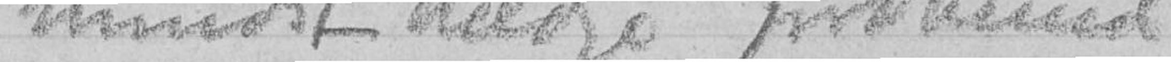mindst heldige grobund
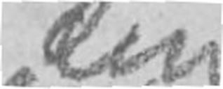den
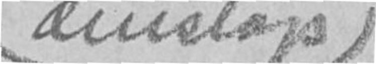denslags
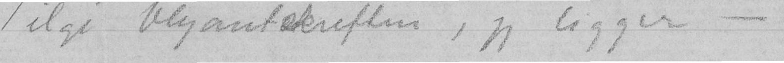Tilgi blyantskriften, jeg ligger -
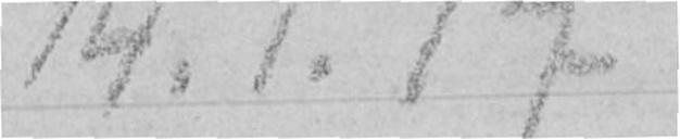14. 1. 17
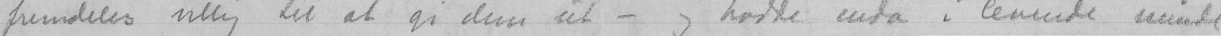fremdeles villig til at gi dem ut - hadde enda i levende minde
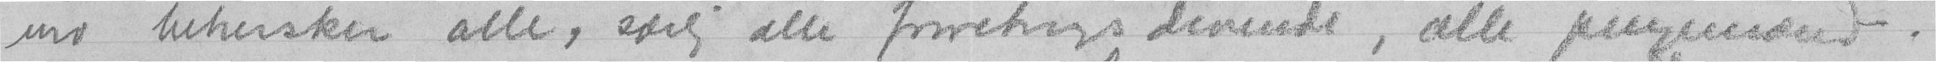uro behersker alle, særlig alle forretningsdrivende, alle pengemænd.
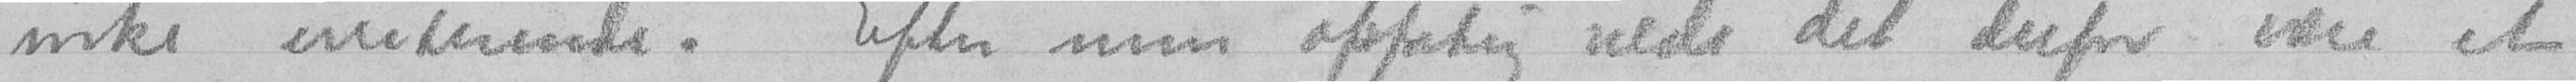virke irriterende. Efter min oppfatning vilde det derfor være et
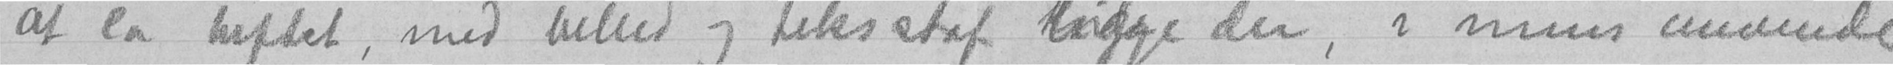at la heftet, med billed og tekststof ligge der, i mens anvende
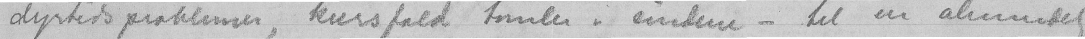dyrtidsproblemer, kursfald tomler i sindene - til en almindelig
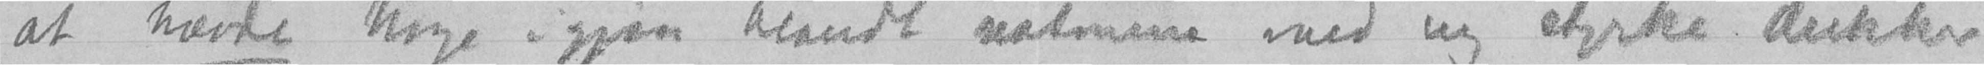at hævde Norge igjen blandt nationene med ny styrke dukker
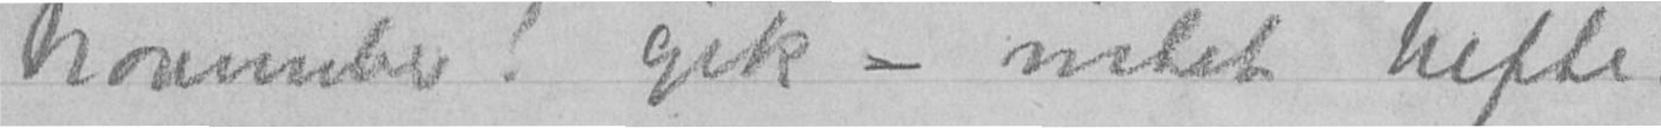November! gik - intet hefte.
